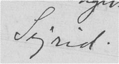Sigrid.
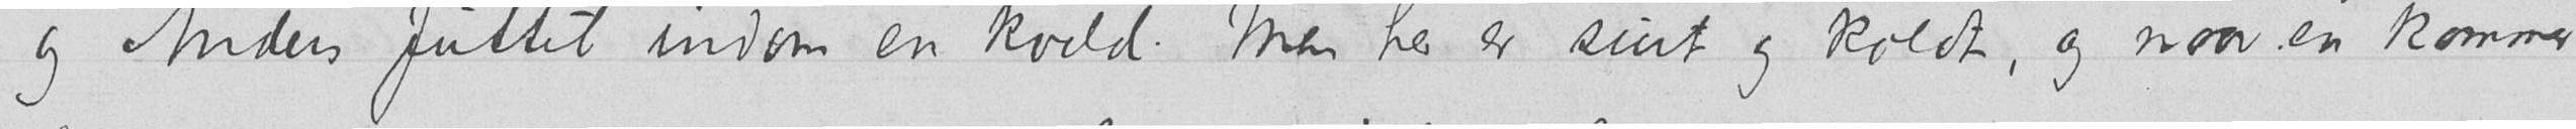og Anders futtet indom en kveld. Men her er surt og koldt, og naar en kommer
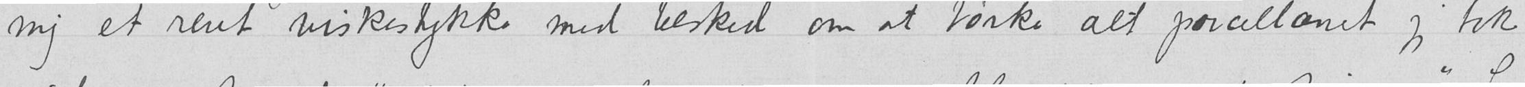mig et rent viskestykke med besked om at tørke alt porcellænet jeg tok
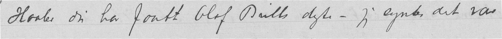Haaber du har faatt Olaf Bulls digte – jeg syntes det var
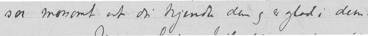saa morsomt at du kjendte dem og er glad i dem.
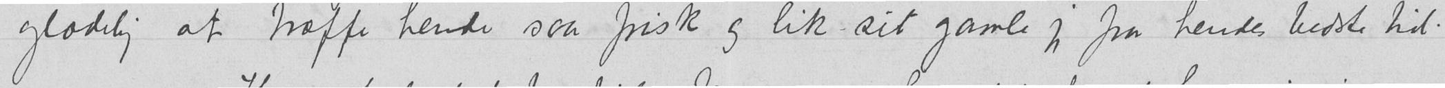glædelig at træffe hende saa frisk og lik sit gamle jeg fra hendes bedste tid.
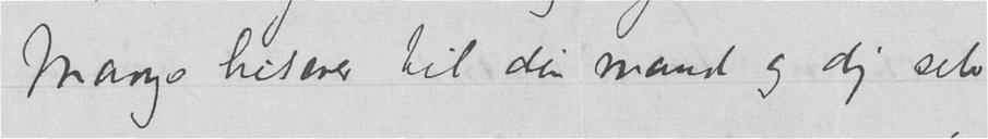Mange hilsener til din mand og dig selv
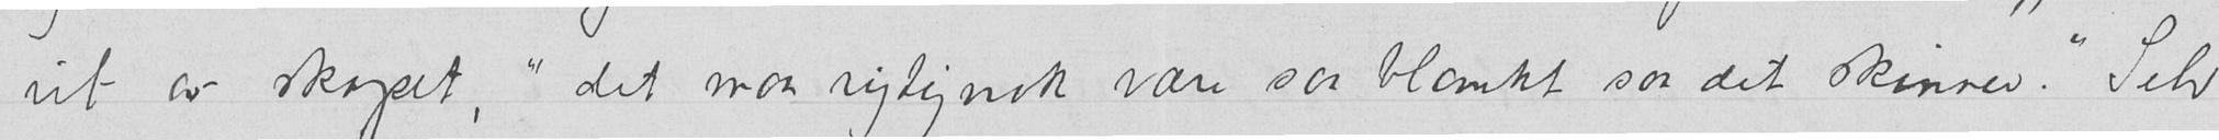ut av skapet, "det maa rigtignok være saa blankt saa det skinner." Selv
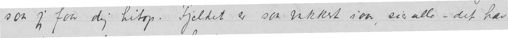saa jeg faar dig hitop. Fjeldet er saa vakkert iaar, sier alle – det har
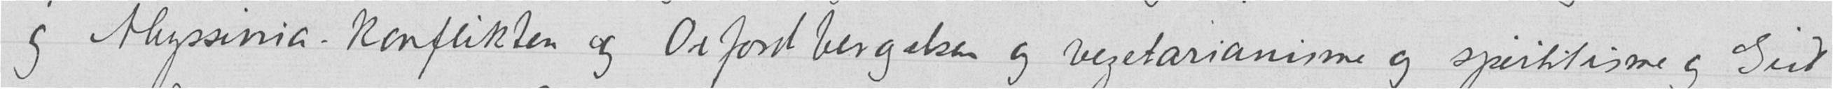og Abyssinia-konflikten og Oxfordbevegelsen og vegetarianisme og spiritisme og Gud
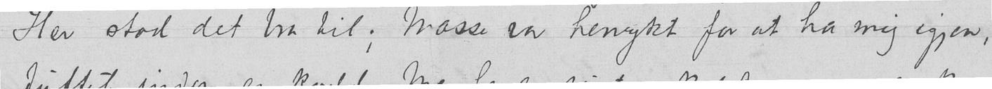Her stod det bra til; Mosse var henrykt for at ha mig igjen,
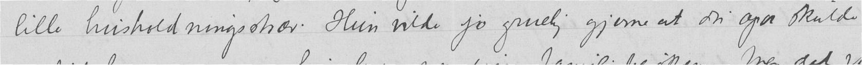lille husholdningsstræv. Hun vilde jo gruelig gjerne at du ogsaa skulde
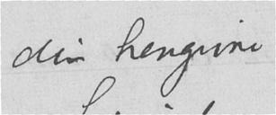din hengivne
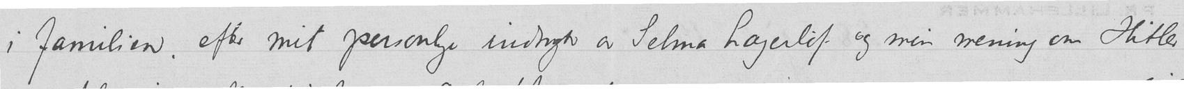i familien, efter mit personlige indtryk av Selma Lagerløf og min mening om Hitler
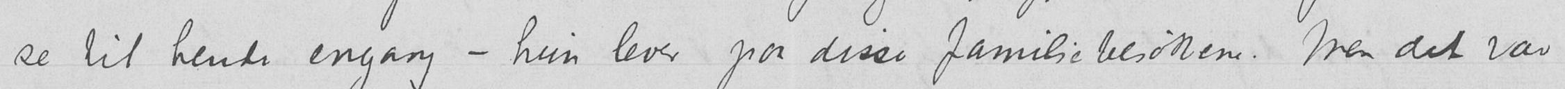se til hende engang – hun lever paa disse familiebesøkene. Men det var
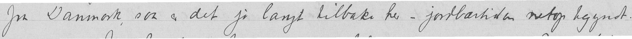fra Danmark, saa er det jo langt tilbake her – jordbærtiden netop begyndt.
